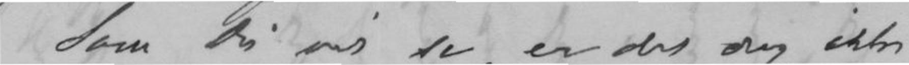Som Du vil se, er det dog ikke
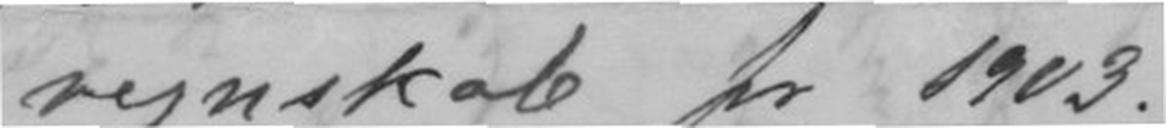regnskab for 1903.
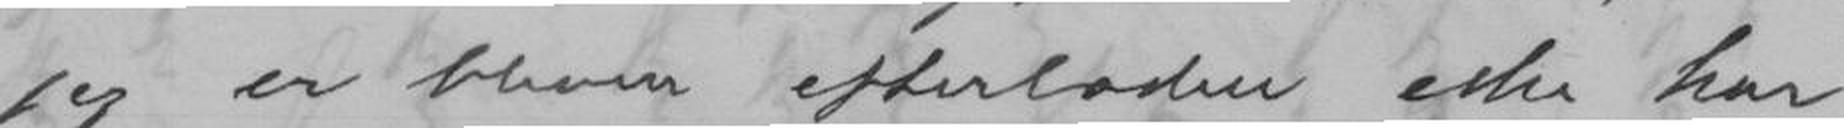jeg er bleven efterladen eller har
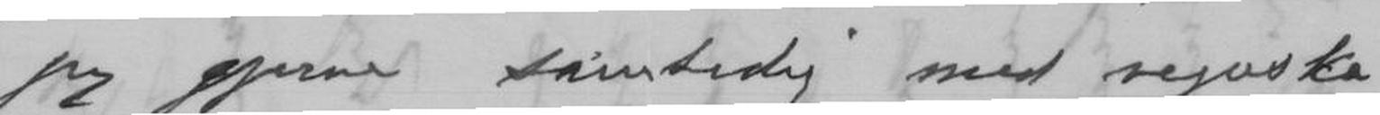jeg gjerne samtidig med regnska-
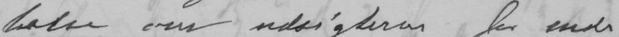lelse over udsigterne for inde-
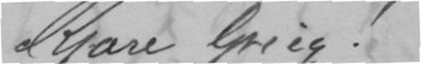Kjære Grieg!
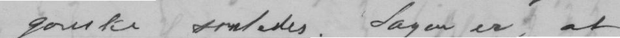ganske saaledes. Sagen er, at
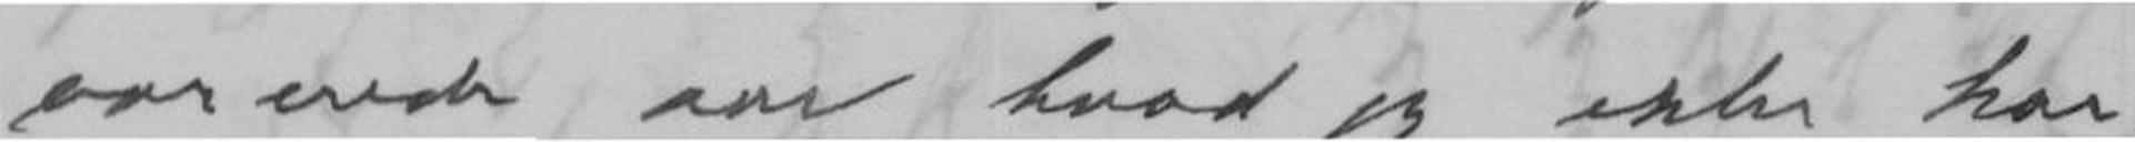værende aar, hvad jeg ikke har
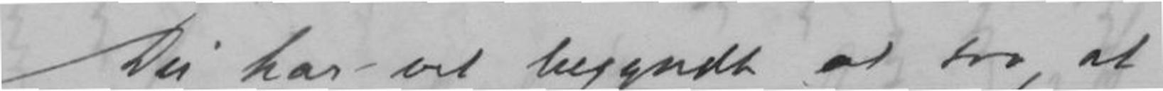Du har vel begynt at tro, at
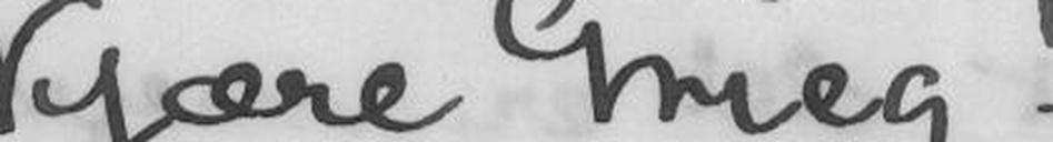Kjære Grieg!
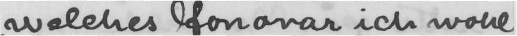welches Honorar ich wohl
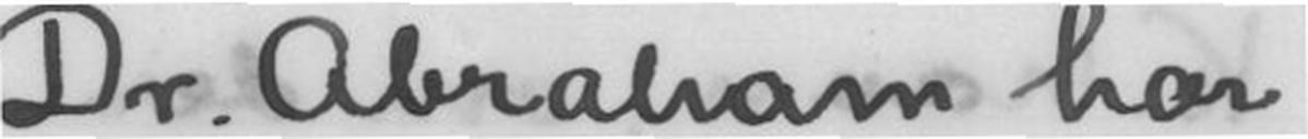Dr. Abraham har
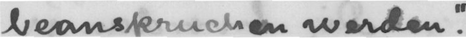beanspruchen werden.
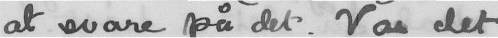at svare på det. Var det
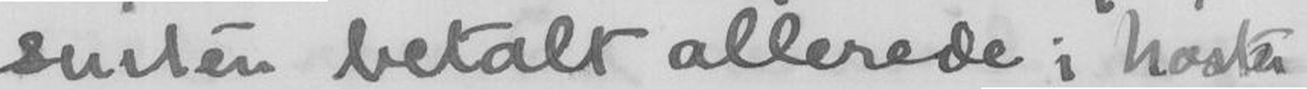suiten betalt allerede i høstes
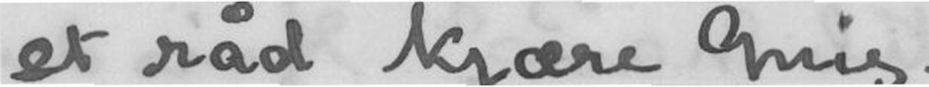et råd kjære Grieg.
